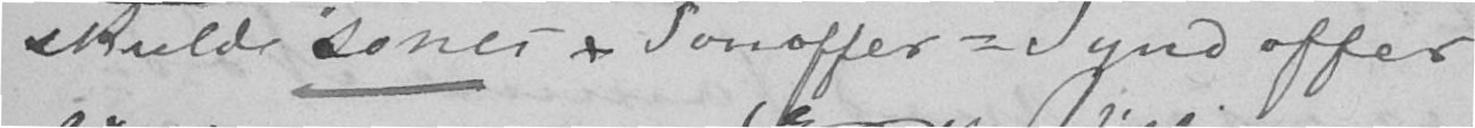skulde sones Sonoffer = Syndoffer –
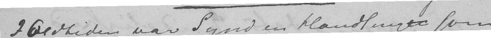I Oldtiden var Synd en Handling som
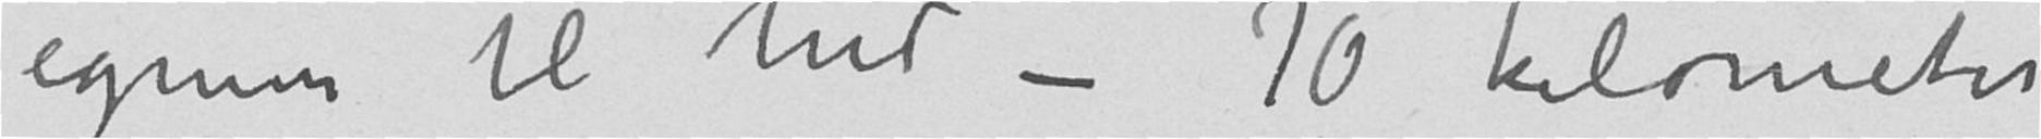egnen til hid – 70 kilometer
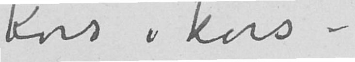Kors i kors –
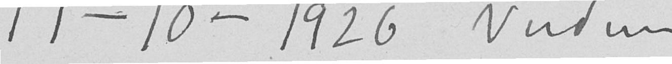17–10–1926 Verdun
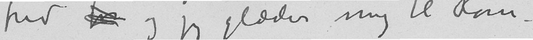fred for og jeg glæder mig til Rom –
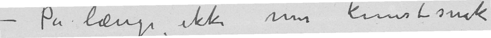– På længe ikke mer kunstsnak
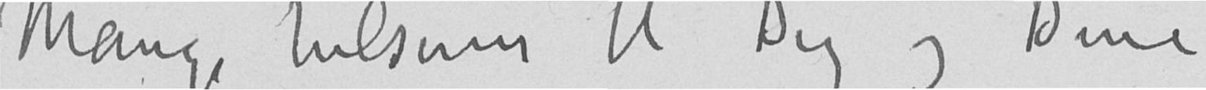Mange hilsener til Dig og Dine
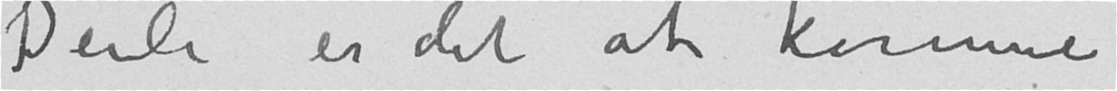Deili er det at komme
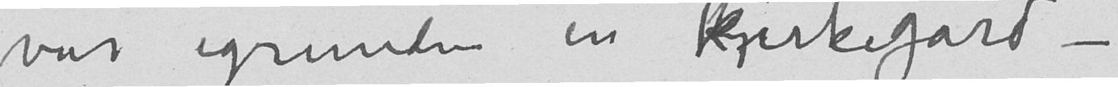var igrunden en kirkegård –
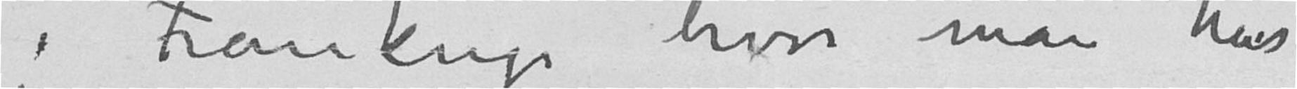i Frankrige hvor man har
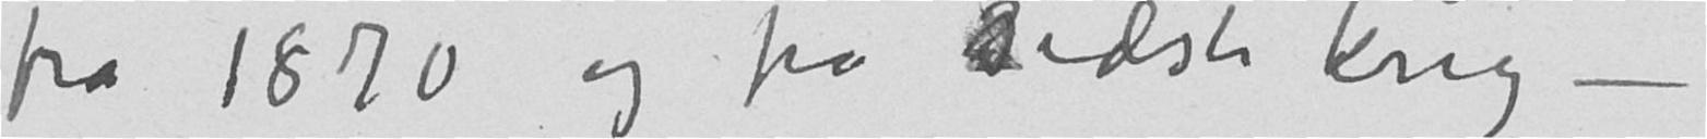fra 1870 og fra sidste krig –
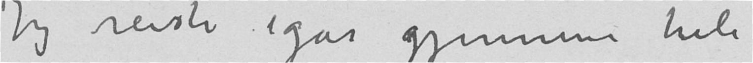Jeg reiste igår gjennem hele
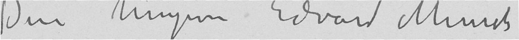Din hengivne Edvard Munch
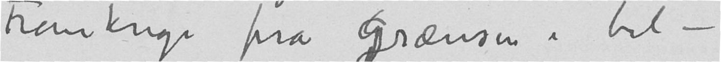Frankrige fra gGrænsen i bil –
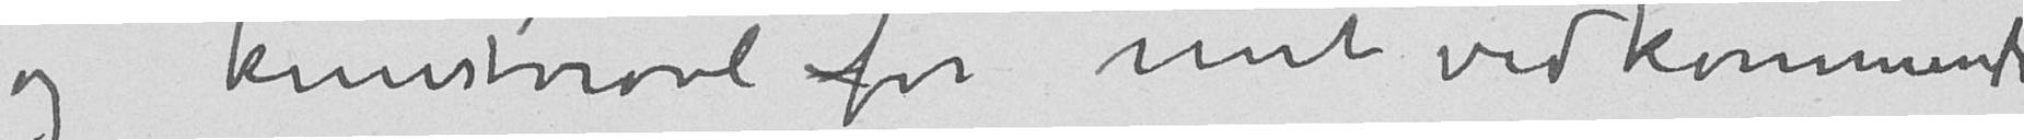og kunstvrøvl for mit vedkommende
